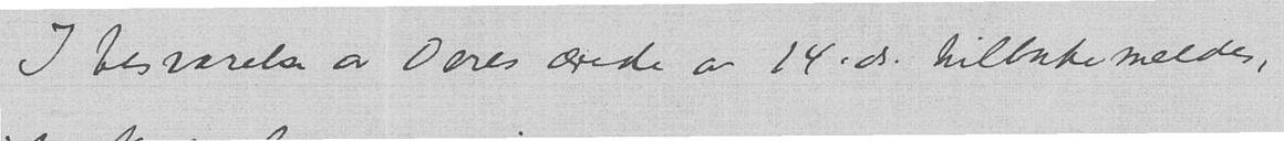I besvarelse av Deres ærede av 14.ds. tilbakemeldes,
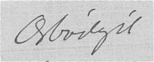Ærbødigst
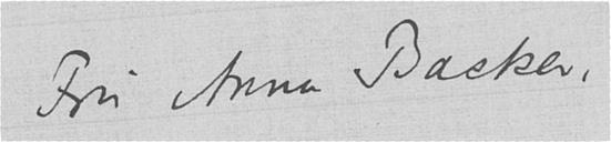Fru Anna Backer,
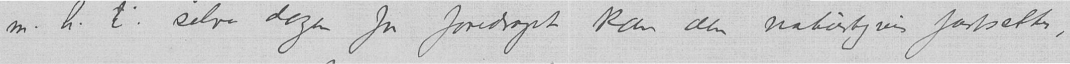m.h.t. selve dagen for foredraget kan den naturligvis fastsettes,
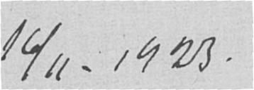16/11-1923.
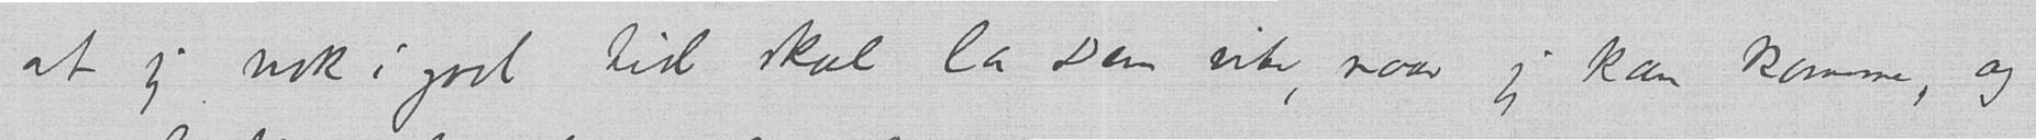at jeg nok i god tid skal la Dem vite, naar jeg kan komme, og
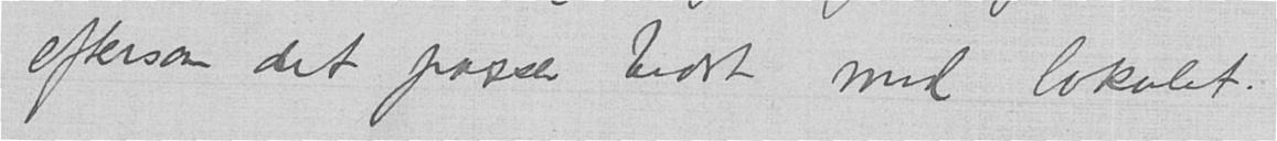eftersom det passer bedst med lokalet.
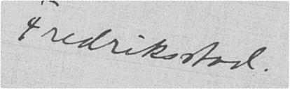Fredriksstad.
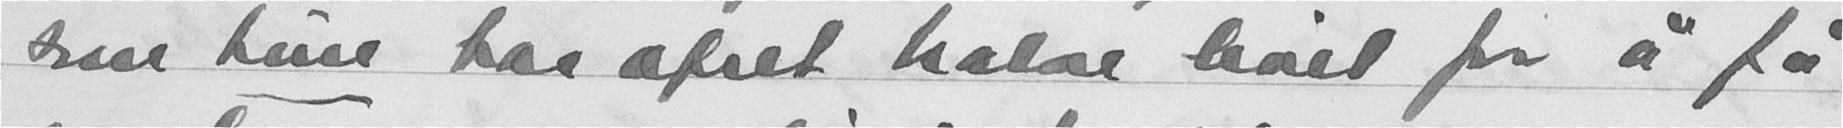som hun har ofret halve livet for å få
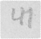1/1
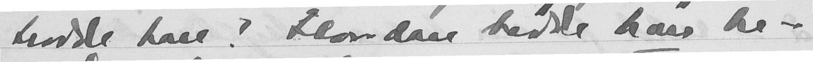trodde han? Hvordan hadde han be-
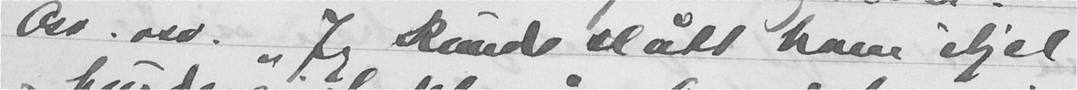Oso. osv. „Jeg kunde slått ham ihjel
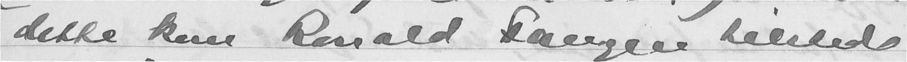dette kom Ronald Fangen tilstede
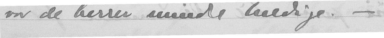var de herrer mindre heldige. -
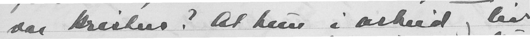var kristen? At hun i arbeid og liv
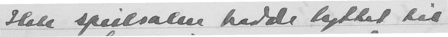Hele speilsalen hadde lyttet til
